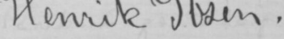Henrik Ibsen.
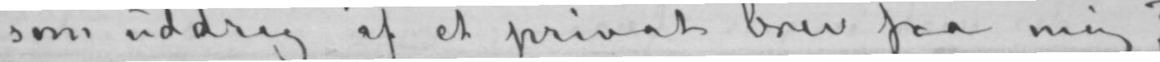som uddrag af et privat brev fra mig?
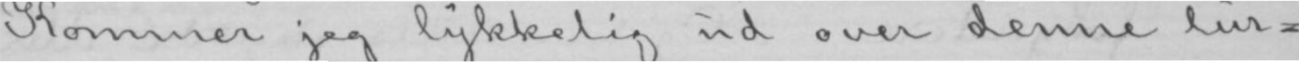Kommer jeg lykkelig ud over denne lur-
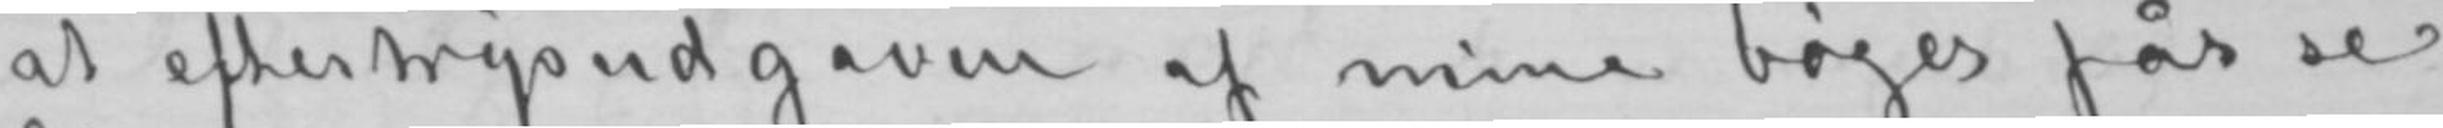at eftertrysudgaven af mine bøger får se
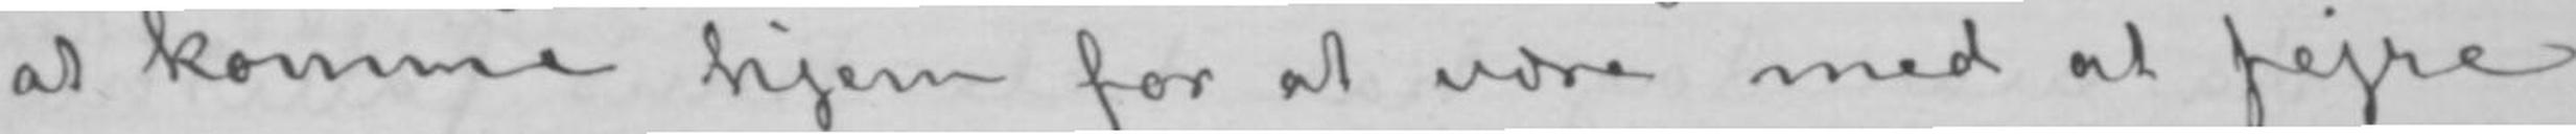at komme hjem for at være med at fejre
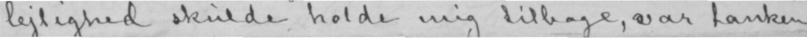lejlighed skulde holde mig tilbage, var tanken
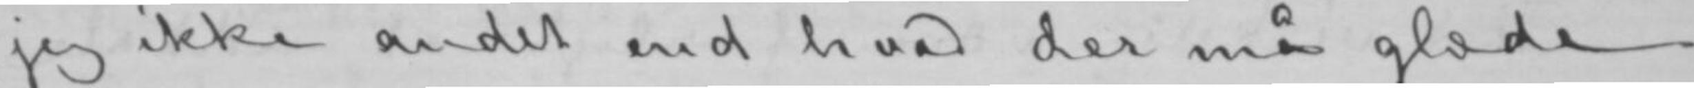jeg ikke andet end hvad der må glæde
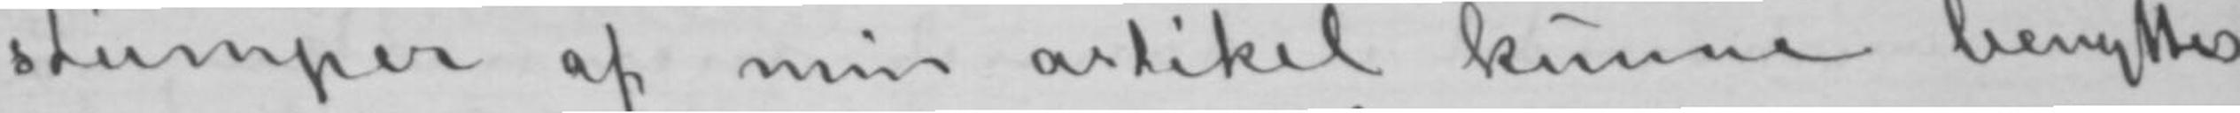stumper af min artikel kunne benyttes
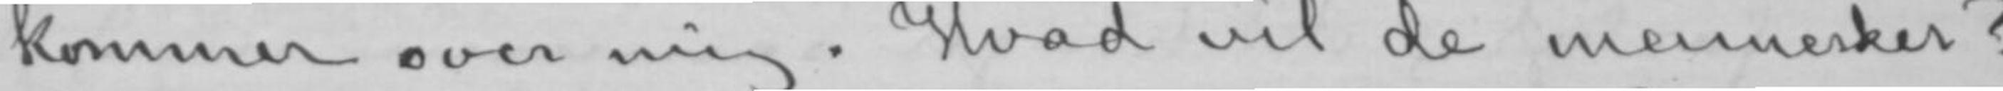kommer over mig. Hvad vil de mennesker?
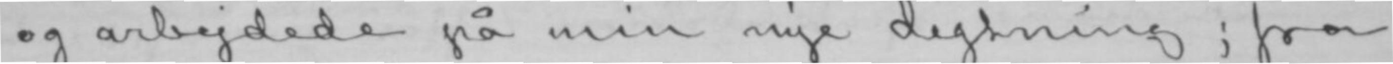og arbejdede på min nye digtning; fra
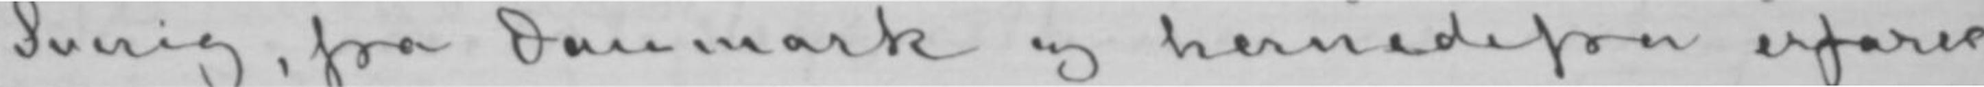Sverig, fra Danmark og hernedefra erfarer
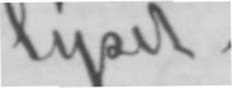lyset.
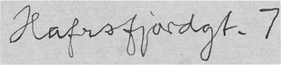Hafrsfjordgt. 7
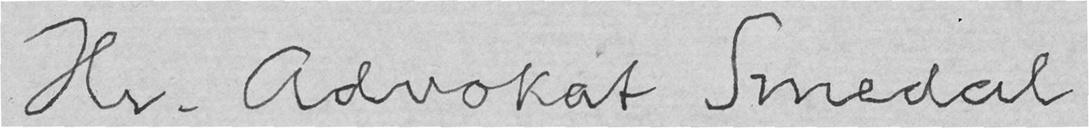Hr. Advokat Smedal
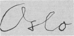Oslo
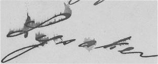Lysaker
